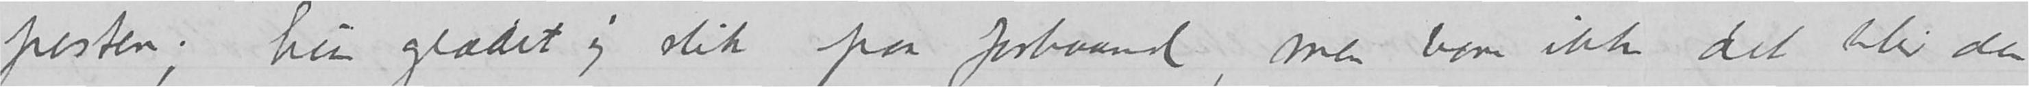posten; hun glædet sig slik paa forhaand, men bare ikke det blir den
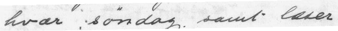hvær søndag, samt læser
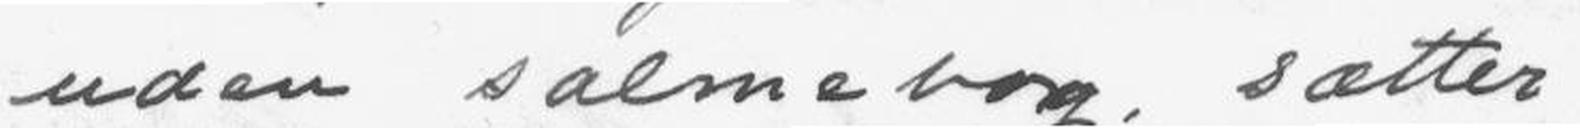uden salmebog, sætter
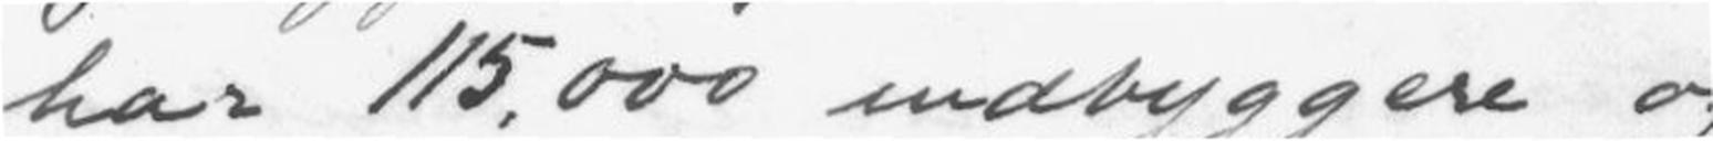har 115,000 indbyggere og
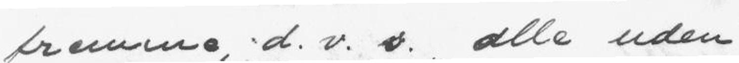fremme, d.v.s. alle uden
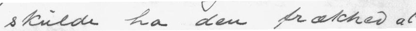skulde ha den frækhed at
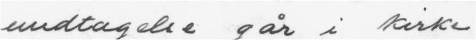undtagelse går i kirke
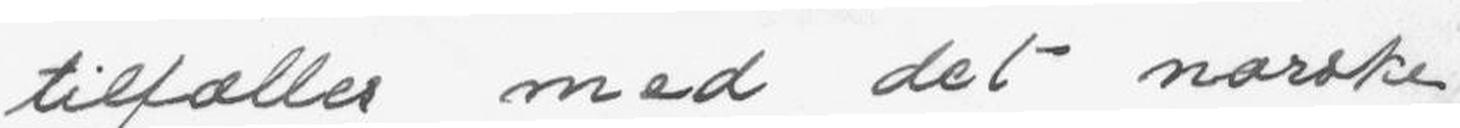tilfælles med det norske
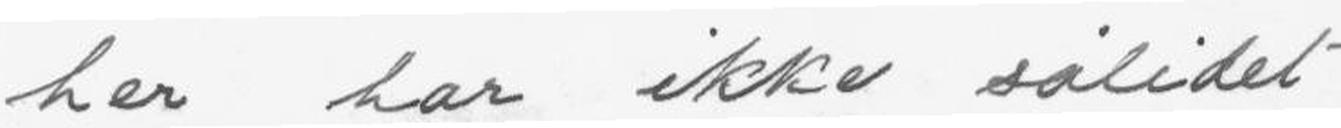her har ikke sålidet
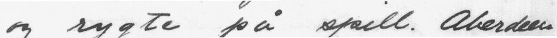og rygte på spill. Aberdeen
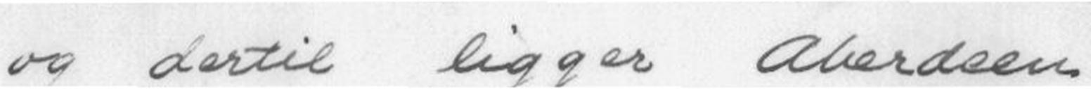og dertil ligger Aberdeen
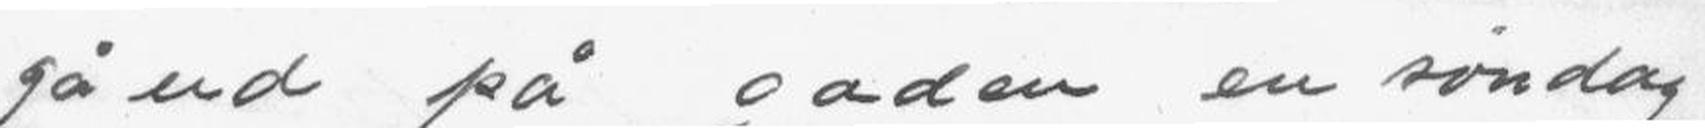gå ud på gaden en søndag
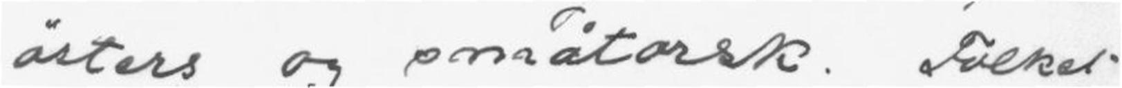østers kog småtorsk. Folket
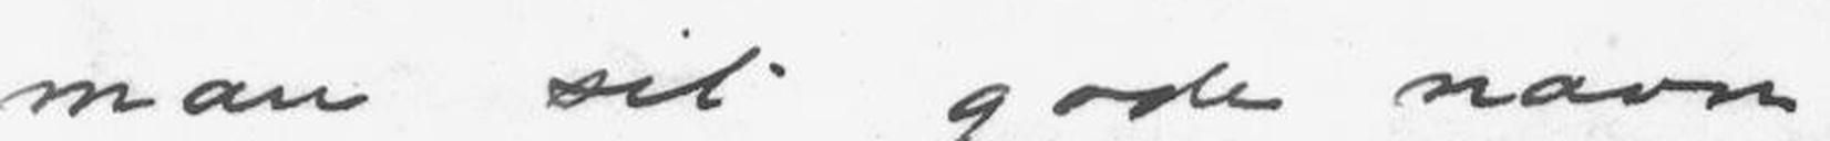man sit gode navn
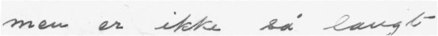men er ikke så langt
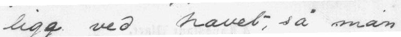lige ved havet, så man
